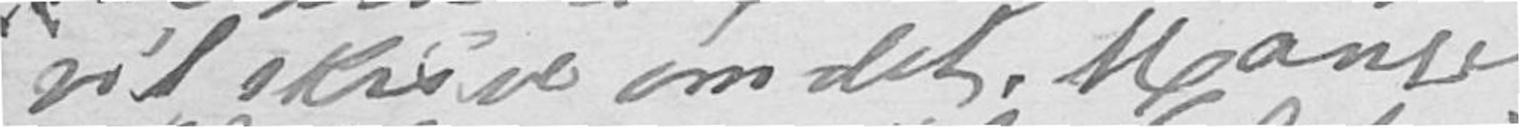vil skrive om det. Mange
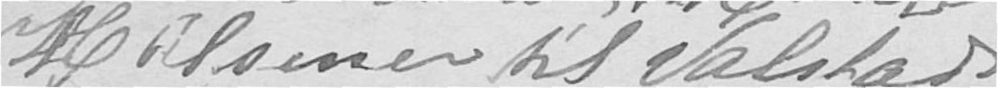Hilsener til Valstads
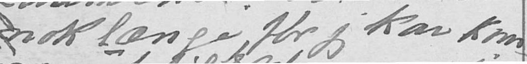nok længe, før jeg kan kom-
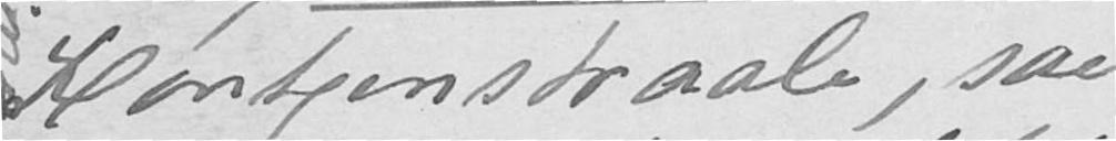Røntgenstraale, saa
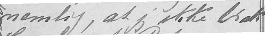nemlig, at jeg ikke brak
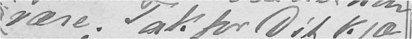være. Tak for Dit kjær
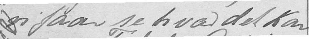vi faar se hvad det kan
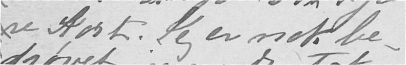re Kort. Jeg er nok be-
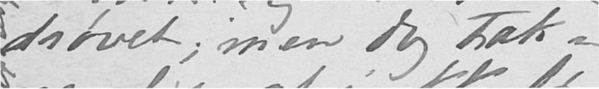drøvet; men dog tak-
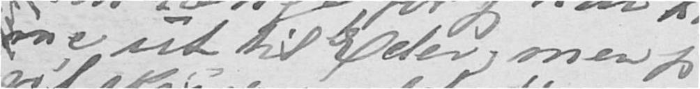me ud til Eder; men jeg
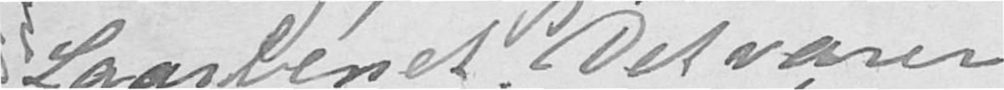Laarbenet. Det varer
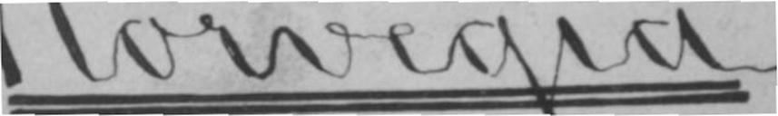(Norvegia.)
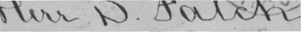Herr S. Falch,
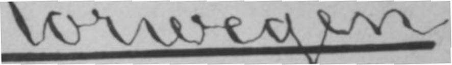Norwegen.
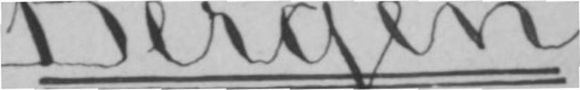Bergen
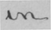in
